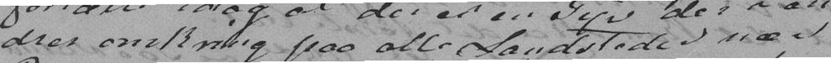drer omkring paa alle Landsteder nær
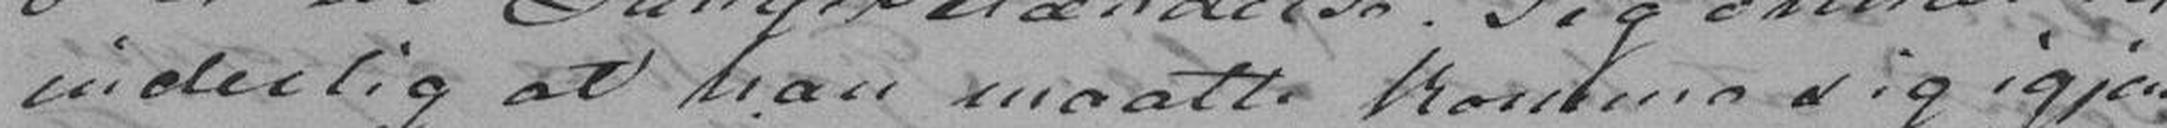inderlig at han maatte komme sig igjen,
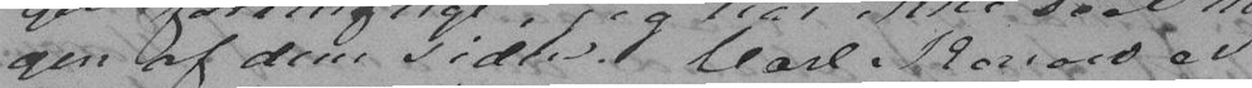gen af dem siden. Carl Konow er
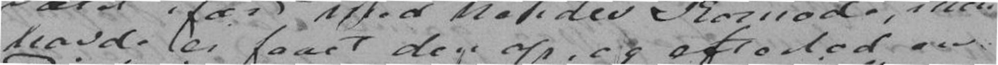havde ei faaet den op, og efterlod en
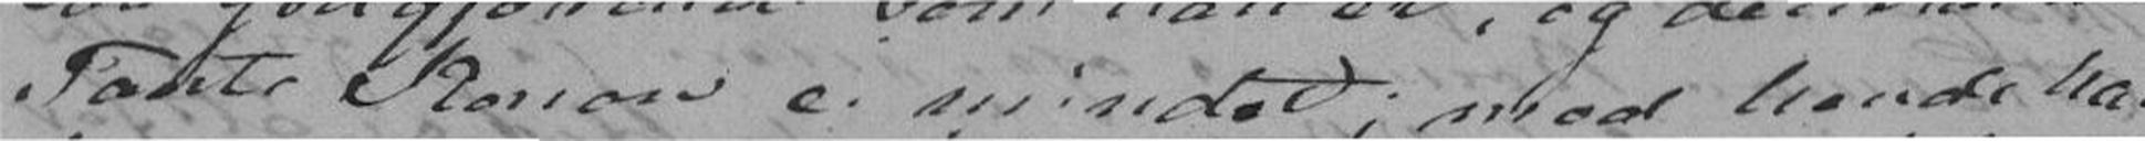Tante Konow ei mindst; mod hende har
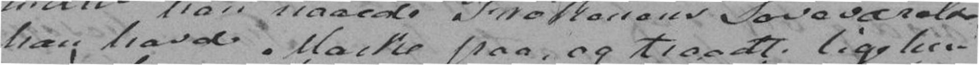han havde Maske paa, og traadte lige hen
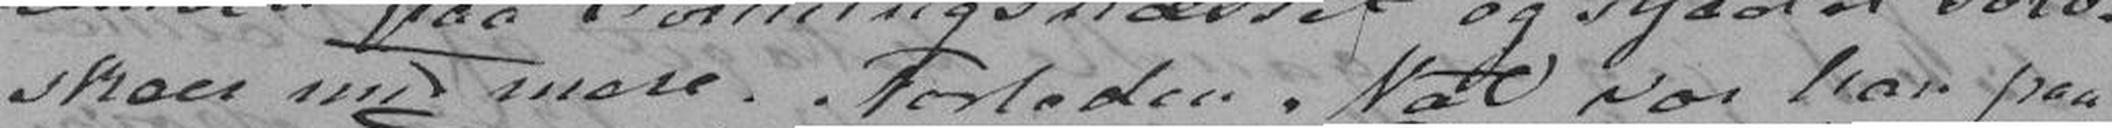skeer med mere. Forleden Nat var han paa
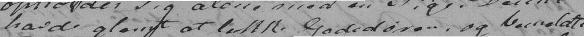havde glemt at lukke Gadedøren, og bemeldte
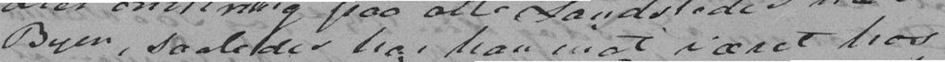Byen, saaledes har han inat været hos
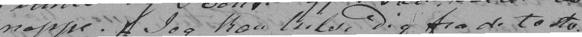neppe. Jeg kan hilse Dig fra de to sto-
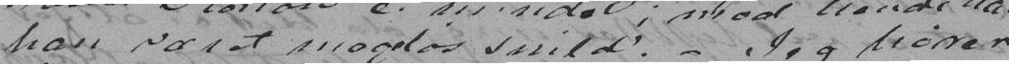hanværet mageløs snild. - Jeg hører
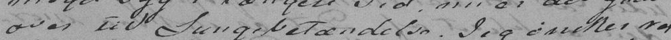over til Lungebetændelse. Jeg ønsker ret
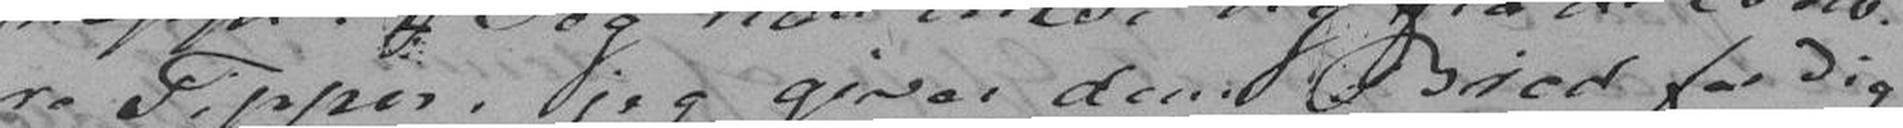re Tipper, jeg giver dem Brød for Dig
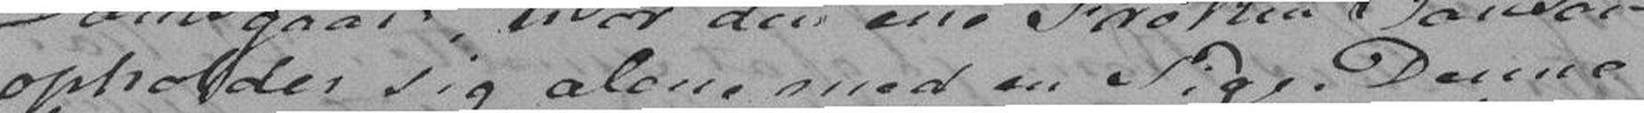opholder sig alene med en Pige. Denne
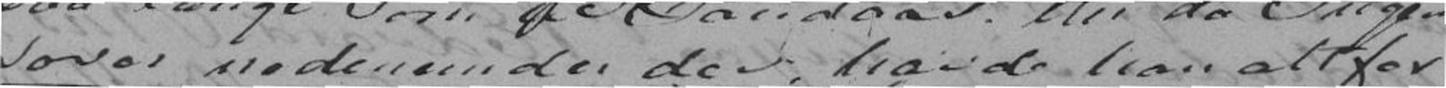sover nedunder der, havde han altfor
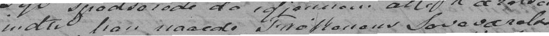indtil han naaede Frøkenens Soveværelse
